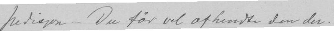pedisjon - Du får vel afhendte den der.
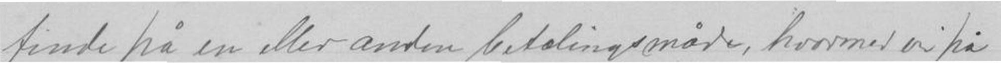finde på en eller anden betalingsmåde, hvormed vi på
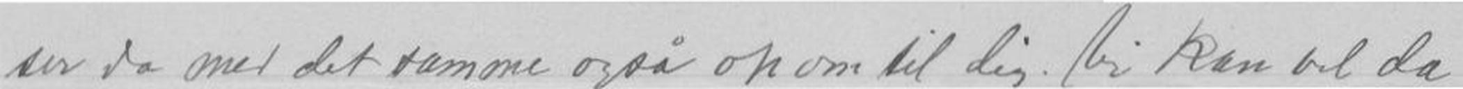ser da med det samme også opom til dig. Vi kan vel da
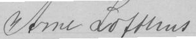Arne Lofthus
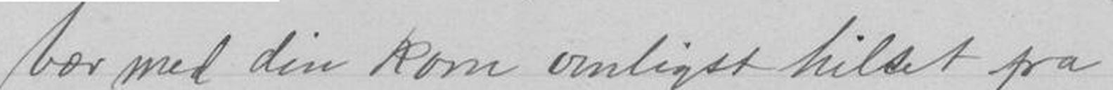Vær med din Kone venligst hilset fra
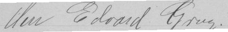Herr Edvard Grieg.
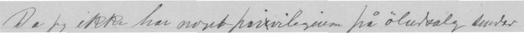Da jeg ikke har noget privilegium på öludsalg sender
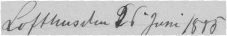Lofthus den 26 juni 1885
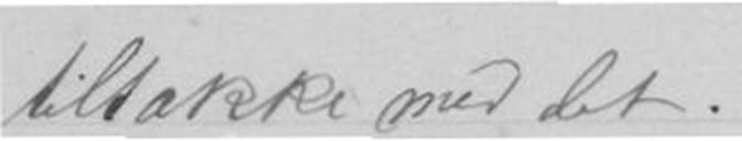tiltakke med det.
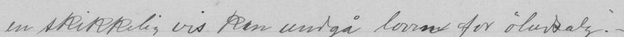en skikkelig vis kan undgå loven for öludsalg.-
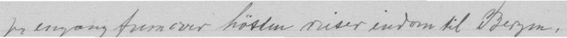jeg engang fremover hösten reiser indom til Bergen,
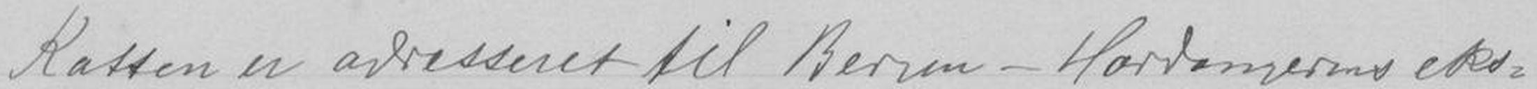Kassen er adresseret til Bergen-Hardangerens eks-
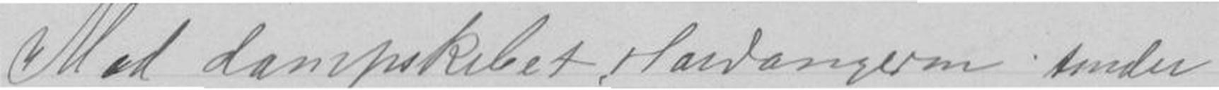Med dampskibet Hardanger sender
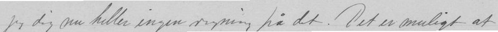jeg dig nu heller ingen regning på det. Det er muligt at
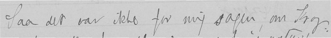Saa det var ikke for mig sagen, om Krog
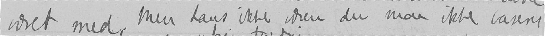været med. Men hans ikke væren du maa ikke bagent
